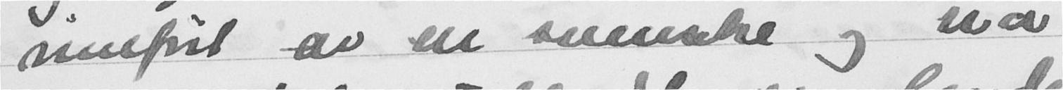innført av en svenske og nå
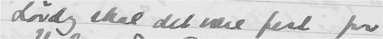Lørdag skal det være fest for
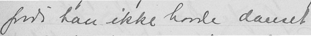fordi han ikke havde danset
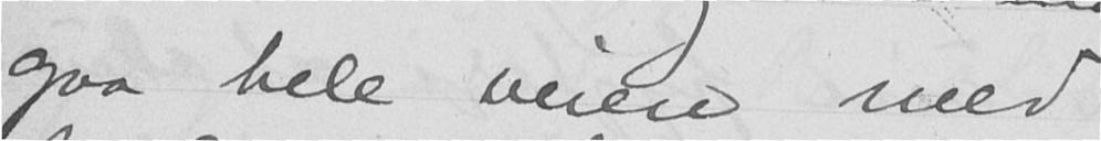gaa hele veien med
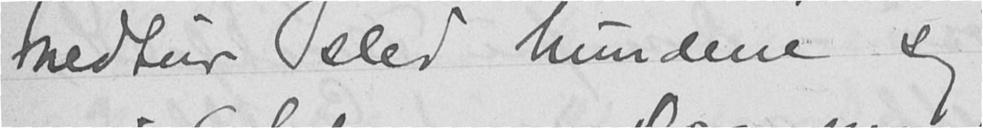nedtur sled hundene sig
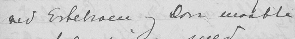ved Ertebroen og Don maatte
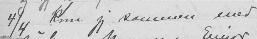4/4 kom jeg sammen med
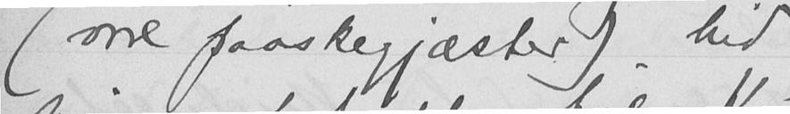(vore paaskegjæster) hid
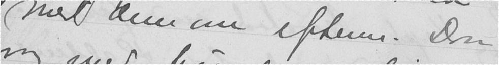med dem om efterm. Don
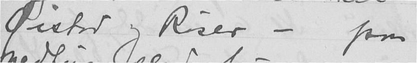Øistad og Røser - paa
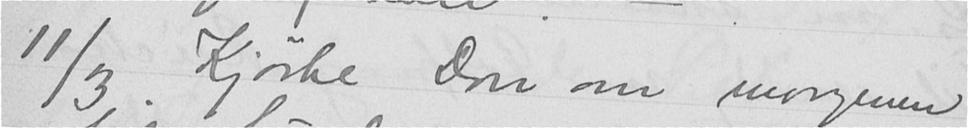11/3 Kjørte Don om morgenen
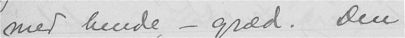med hende - græd. Den
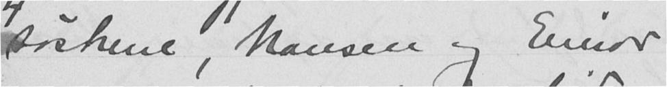søstrene, Nansen og Einar
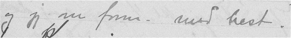og jeg om form. med hest.
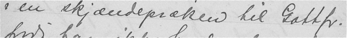i en skjændepræken til Gottfr.
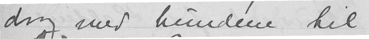drog med hundene til
